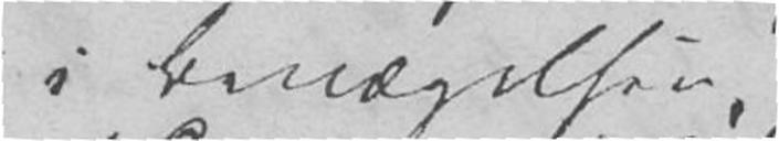i Bevægelsen,
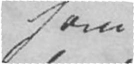som
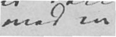med en
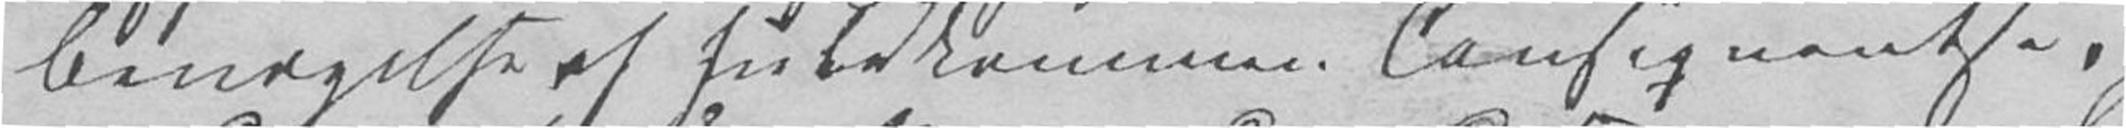Bevægelse af fuldkommen Conseqventse,
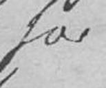for
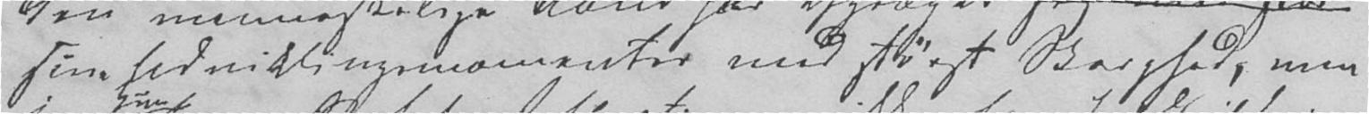sine Udviklingsmomenter med størst Skarphed, men
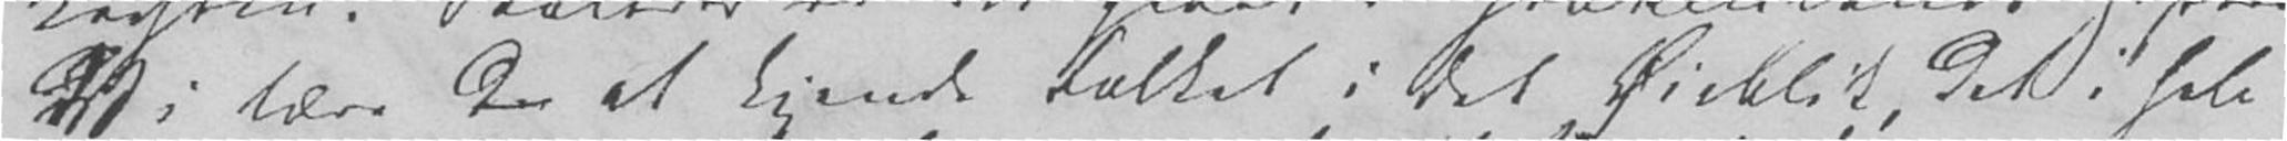Vi lære der at kjende Folket i det Øieblik, det i hele
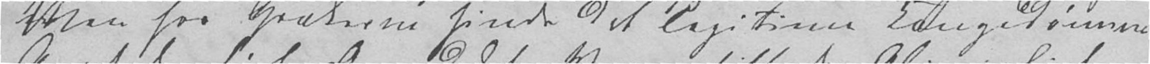Men hos Grækerne hindre det legitime Kongedømme
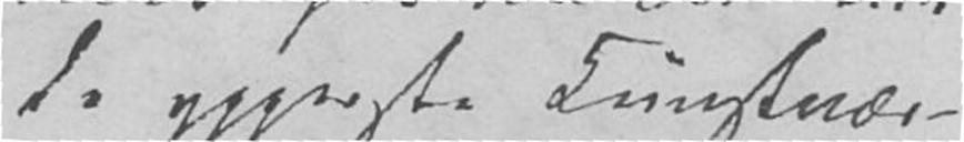de ypperste Kunstvær-
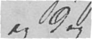og dog
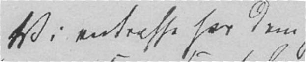Vi antræffe hos dem
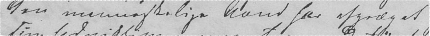den menneskelige Aand har afpræget
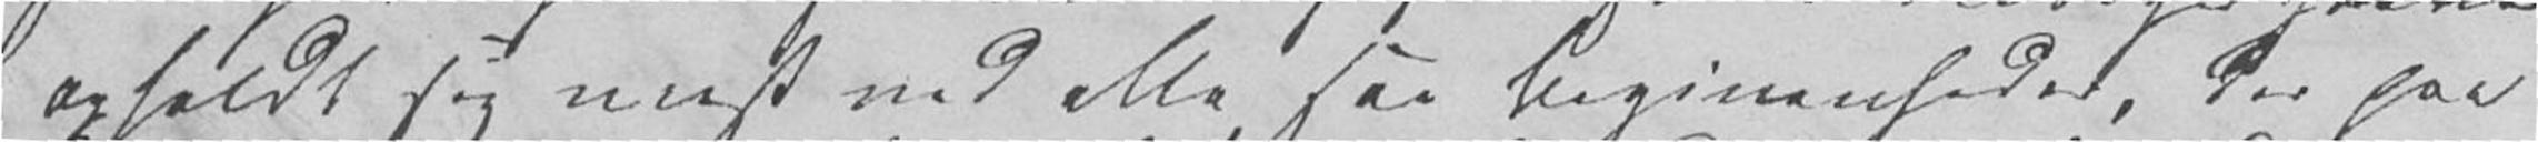opholdt sig meest ved alle saa Begivenheder, der paa
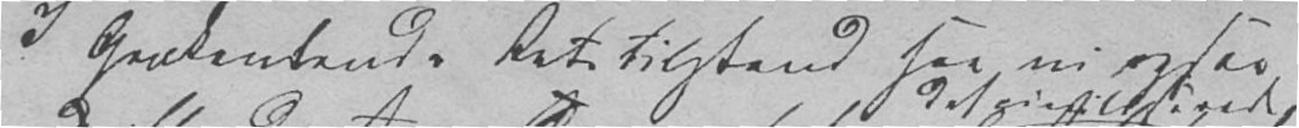I Grækenlands Retstilstand saa vi ogsaa
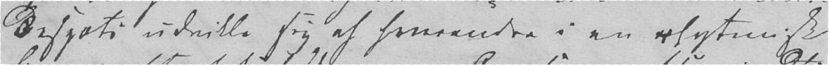Despoti udvikle sig af hverandre i en rhytmisk
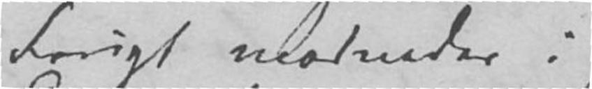først modnedes i
